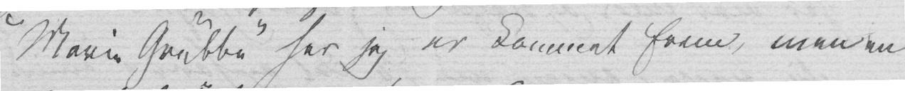"Marie Grubbe" ser jeg er kommet frem, men en
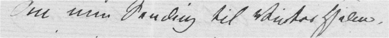Om min Sending til Vinter Hjelm,
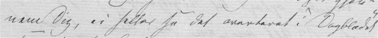nem Dig, ei heller se det averteret i "Dagbladet"
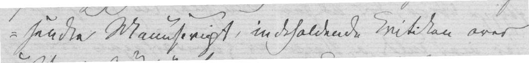sendte Manuscript, indeholdende Kritiken over
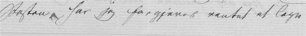Posten, har jeg forgjeves ventet et Tegn
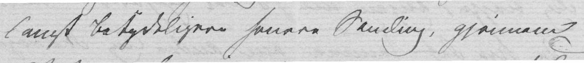langt betydeligere senere Sending, gjennem
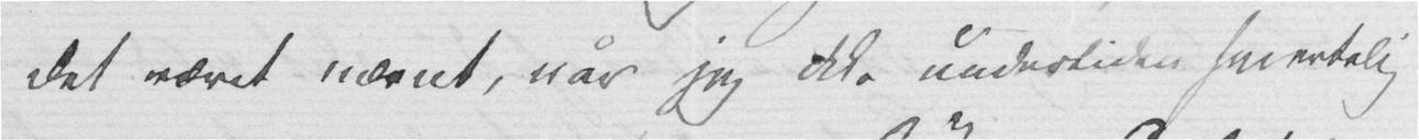det været nævnt, når jeg ikke undertiden smertelig
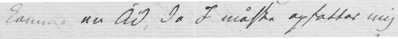komme en Tid, da I måske opfatter mig
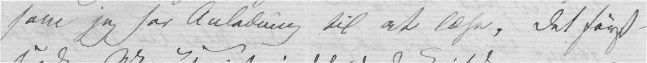som jeg har Anledning til at læse. Det først-
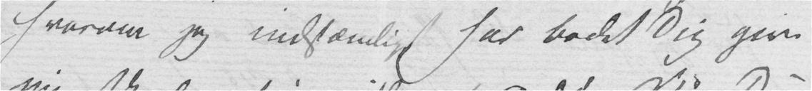hvorom jeg indstændigt har bedet Dig give
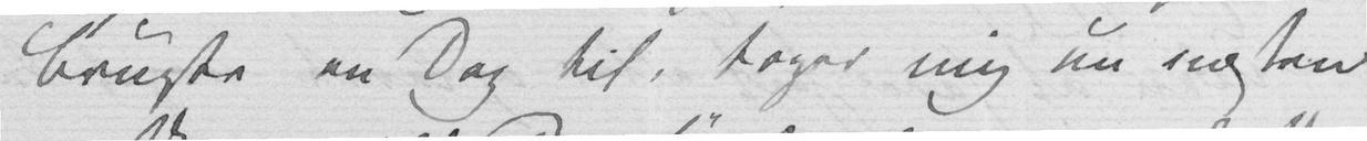brugte en Dag til, tager mig nu næsten
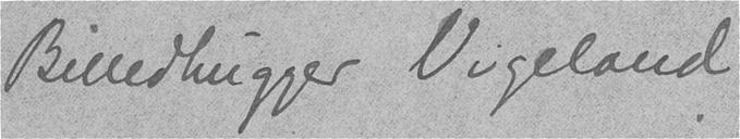Billedhugger Vigeland
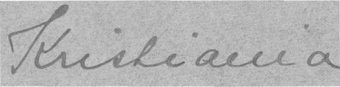Kristiania
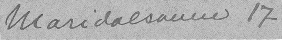Maridalsveien 17
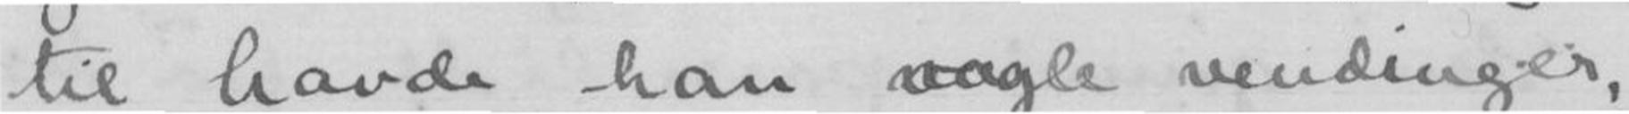til havde han nogle vendinger,
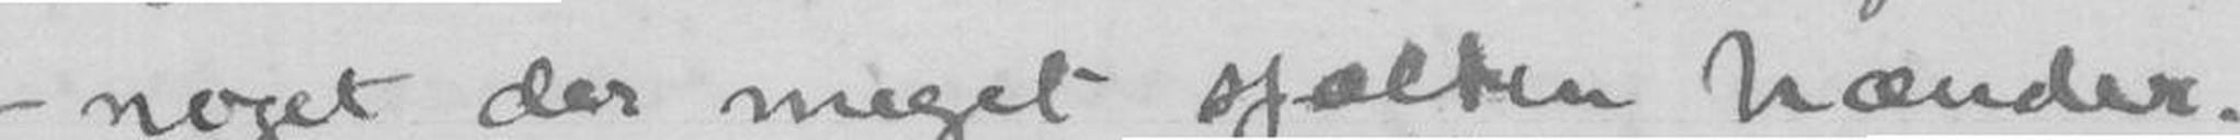- noget der meget sjelden hænder.
